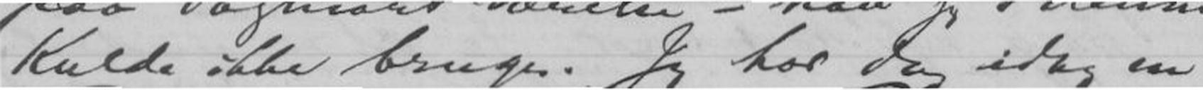Kulde ikke bruge. Jeg har dog idag en
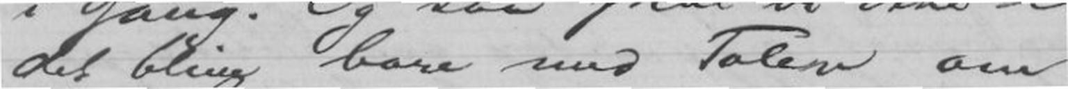det blive bare med Talen om
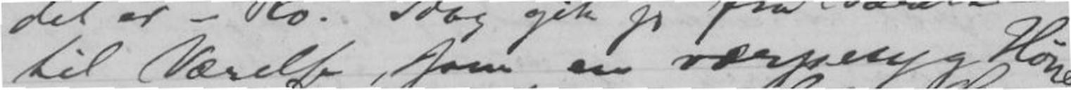til Værelse, som en værpesyg Høne,
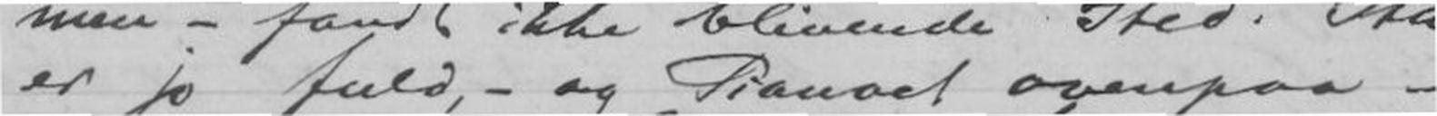er jo fuld, - og Pianoet ovenpaa -
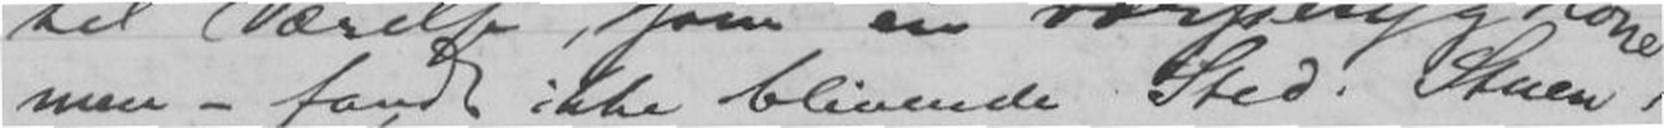men - fandt ikke blivende Sted. Stuen
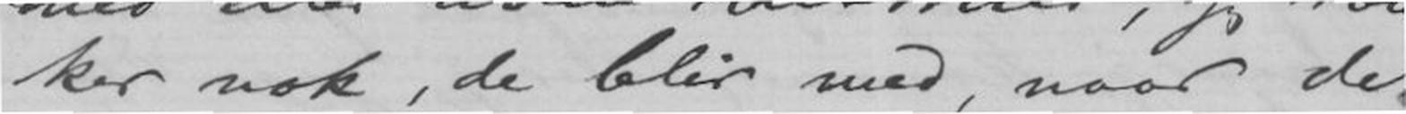ker nok, de blir med, naar det
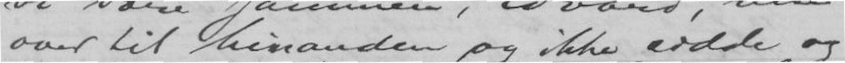over til hinanden og ikke sidde
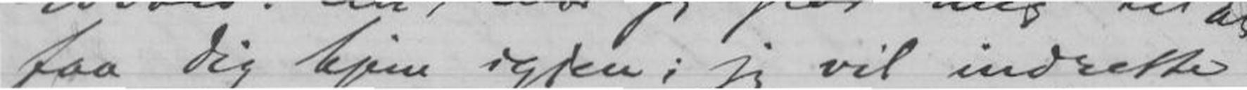faa Dig hjem igjen; jeg vil indrette
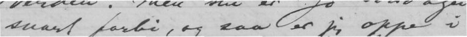snart forbi, og saa er jeg oppe i
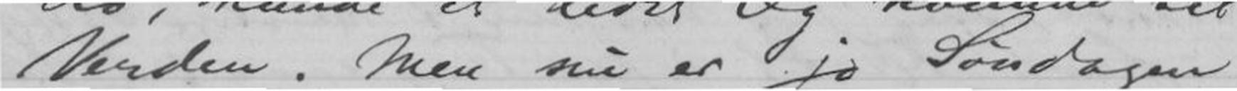Verden. Men nu er jo Søndagen
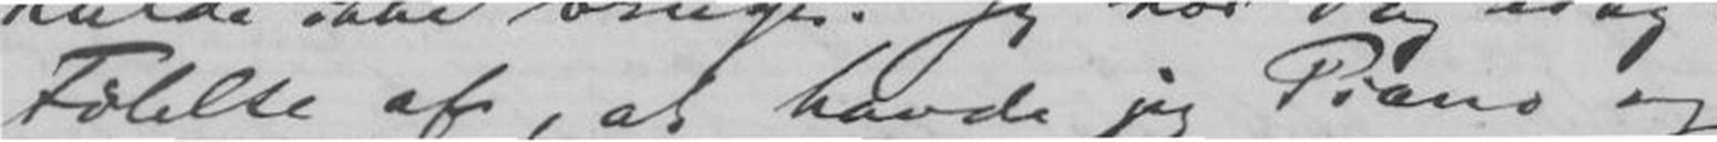Følelse af, at havde jeg Piano og
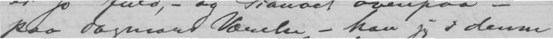paa Dagmars Værelse - kan jeg i denne
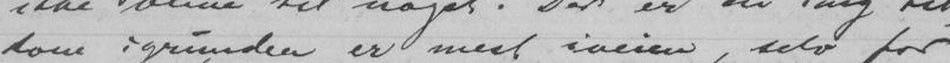som igrunden er mest iveien, selv for
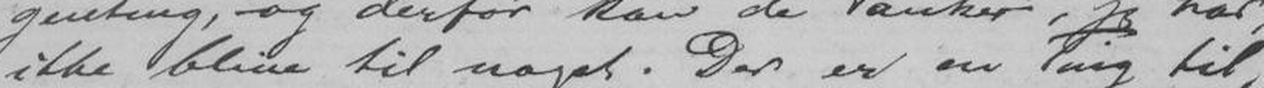ikke blive til noget. Der er en Ting til,
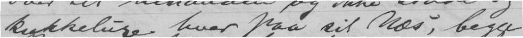og kukkelure hver paa sit Næs, begge
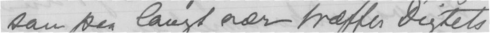og som paa langt nær træffer Digtets
